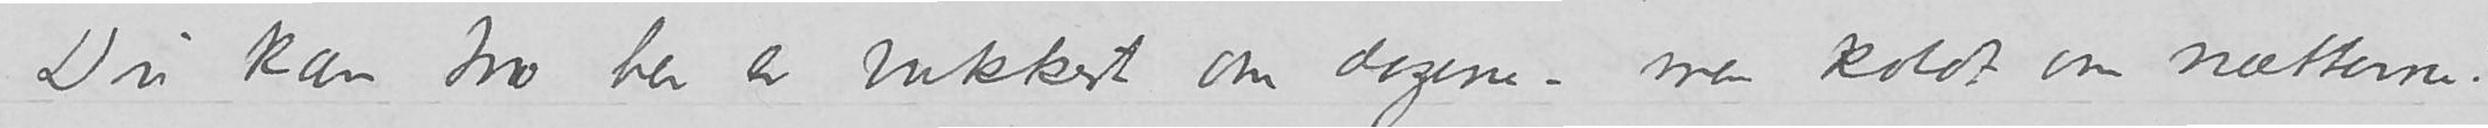Du kan tro her er vakkert om dagen - men koldt om nætterne!
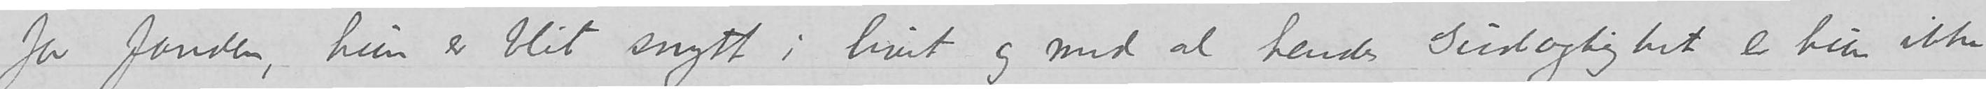for fanden, hun er blit snytt i livet og med al hendes Gudagtighet er hun ikke
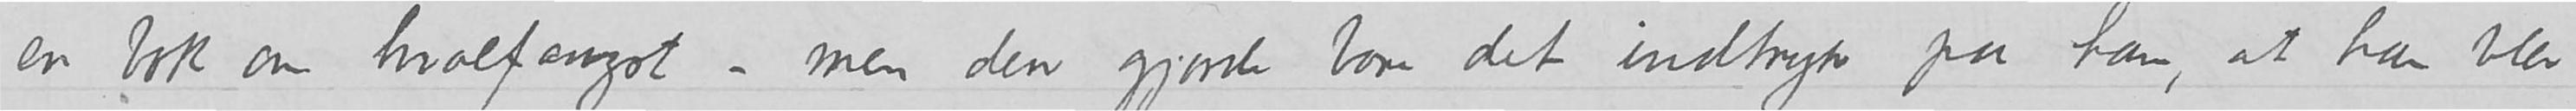en bok om hvalfangst - men den gjorde bare det indtryk paa ham, at han blev
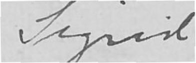Sigrid
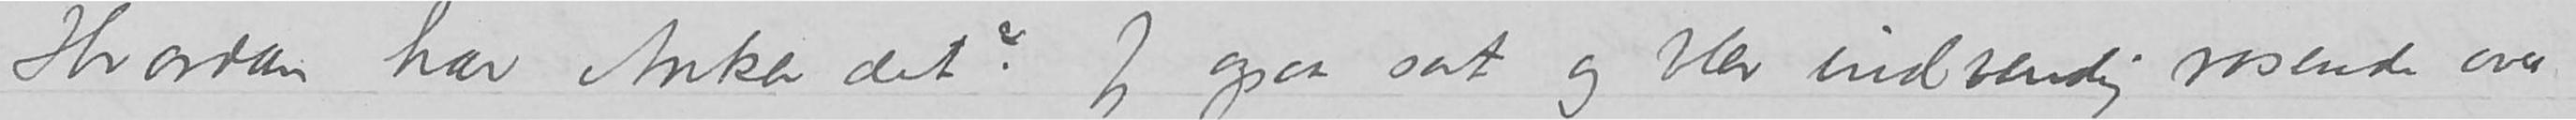Hvordan har Anker det? Jeg ogsaa sat og blev indvendig rasende over
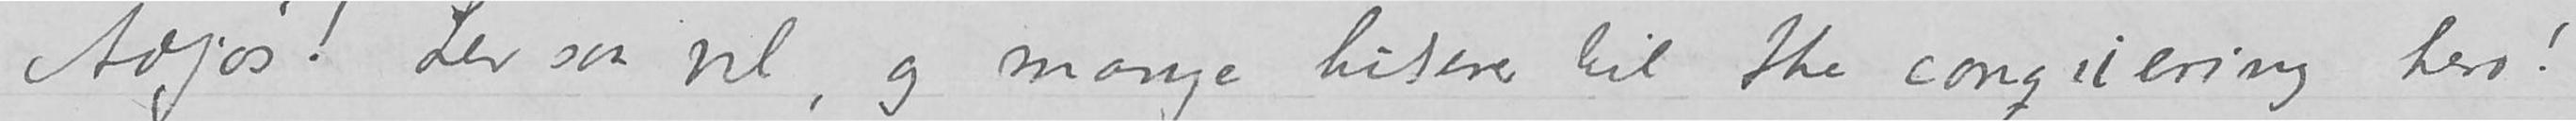Adjøs! Lev saa vel, og mange hilsener til the conqilering hero!
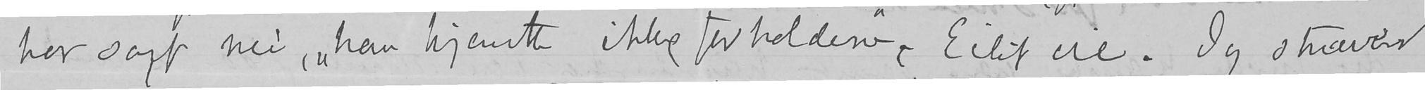har sagt nei, "han kjendte ikke forholdene", Eilif vil. Jeg stræver
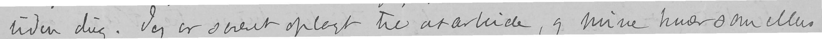uden dug. Jeg er svært oplagt til at arbeide, og mine knær som ellers
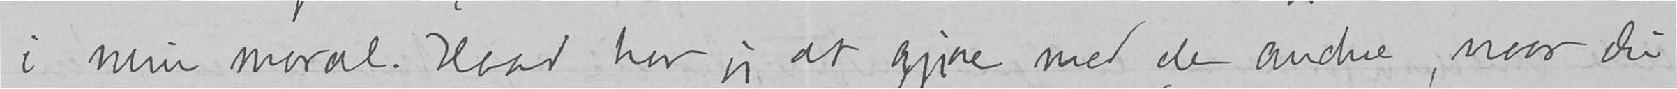i min moral. Hvad har jeg at gjøre med de andre, naar du
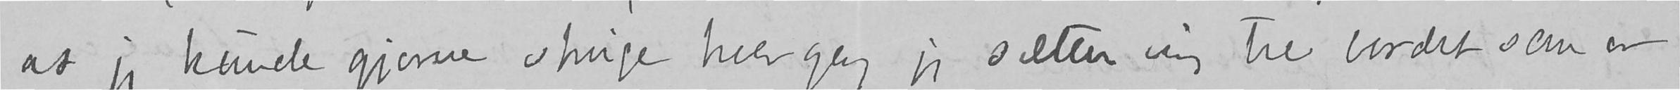at jeg kunde gjerne skrige hver gang jeg sætter mig til bordet som er
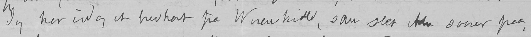Jeg har idag et brevkort fra Werenskiold, som slet ikke svarer paa,
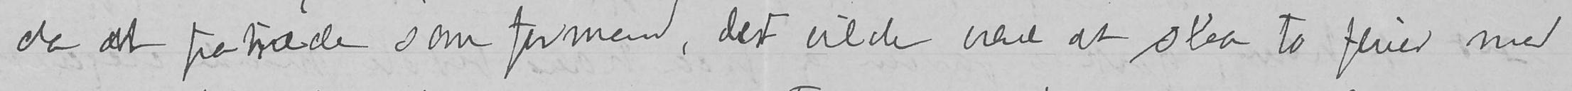da at fratræde som formand, det vilde være at slaa to fluer med
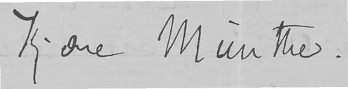Kjære Munthe.
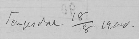Tengesdal 18/8 1900.
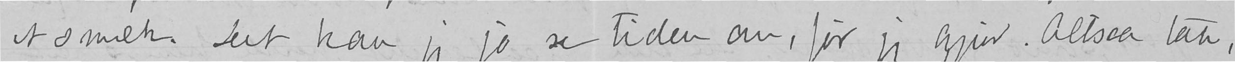et smæk. Det kan jeg jo se tiden om, før jeg gjør. Altsaa tak,
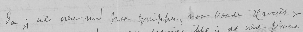Ja jeg vil være med paa gruppen; naar baade Harriet og
